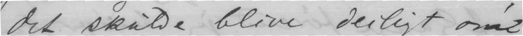det skulde blive deiligt om
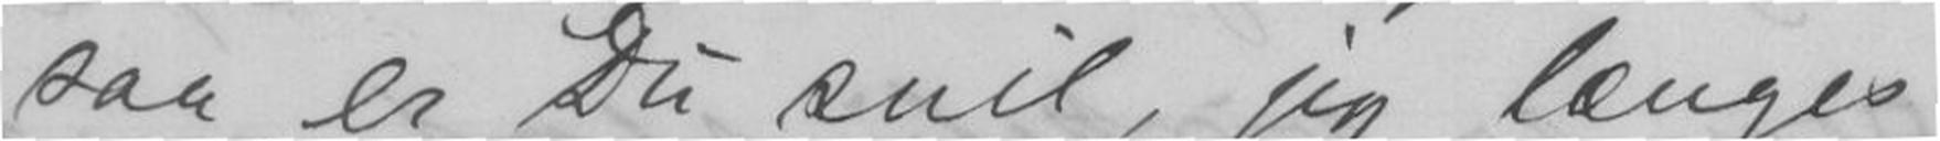saa er Du snil, jeg længes
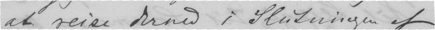at reise derned i Slutningen af
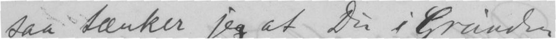saa tænker jeg at Du i grunden
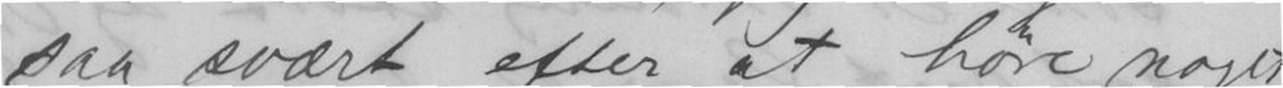saa svært efter at høre noget
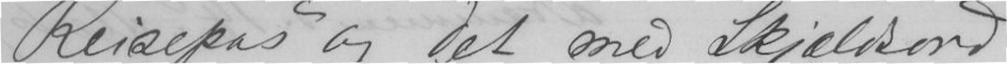Reisepas og det med Skjæsord
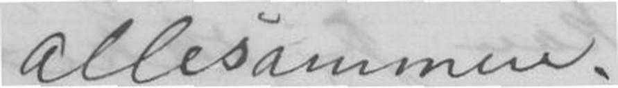allesammen.
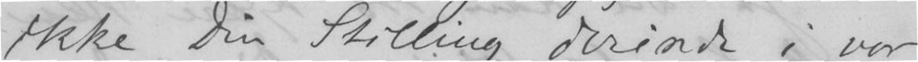ikke Din Stilling derinde i vor
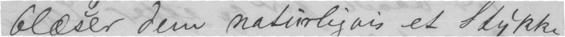blæser dem naturligvis et Stykke
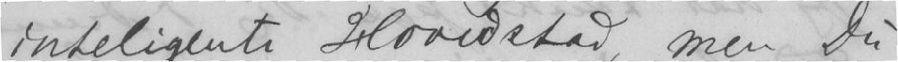interligente Hovedstad, men Du
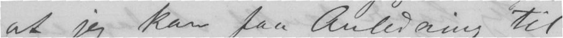at jeg kan faa Anledning til
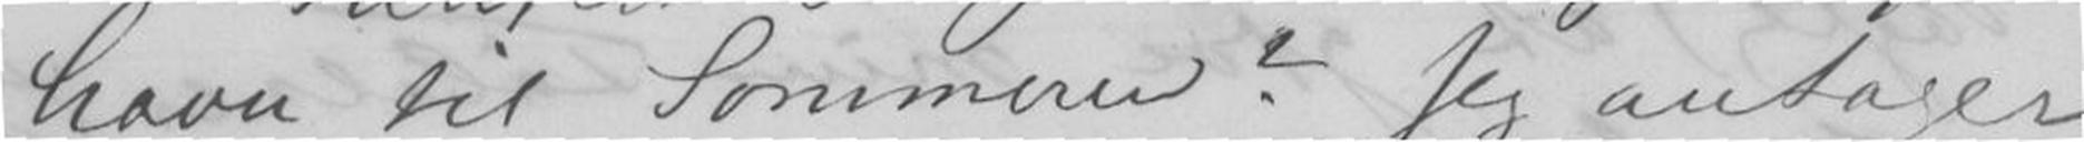havn til Sommeren? Jeg antager
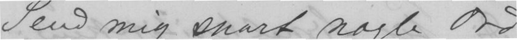Send mig snart nogle Ord
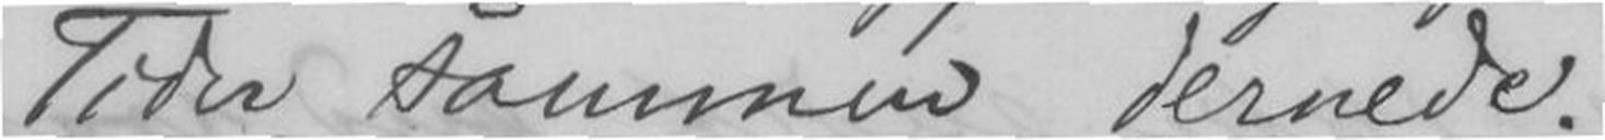Tider sammen dernede.
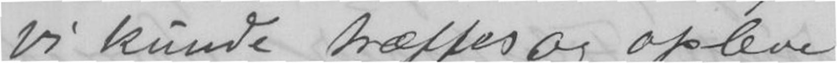vi kunde træffes og opleve
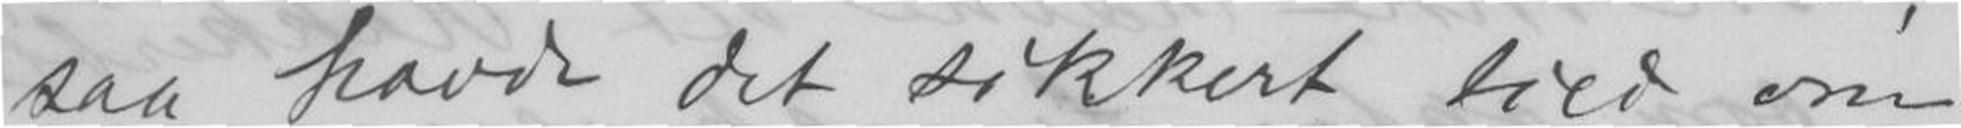saa havde det sikkert tied om
